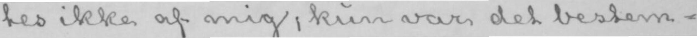tes ikke af mig; kun var det bestem-
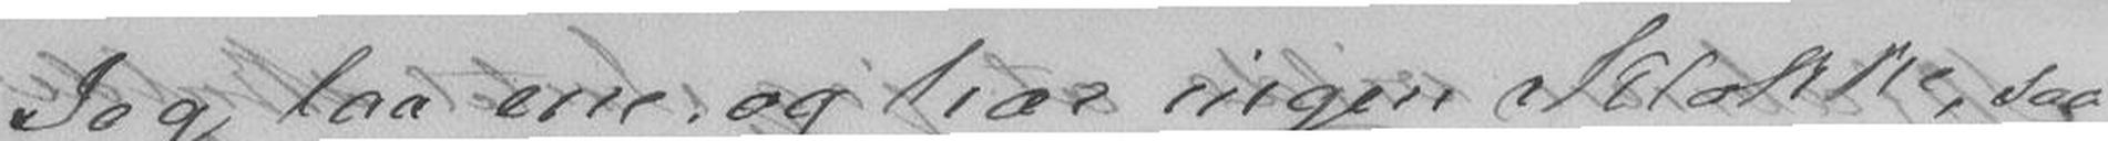Jeg laa ene og ingen Klokke, saa
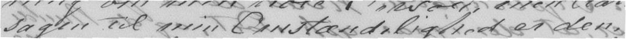sagen til min Omstændelighed er den,
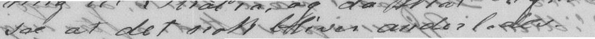see at det nok bliver anderledes.
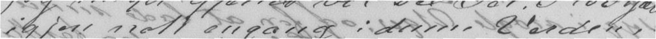igjen nok engang i denne Verden,
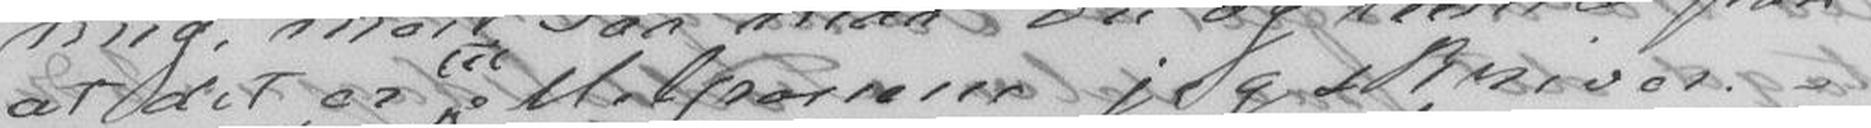at det er til Melponene jeg skriver.
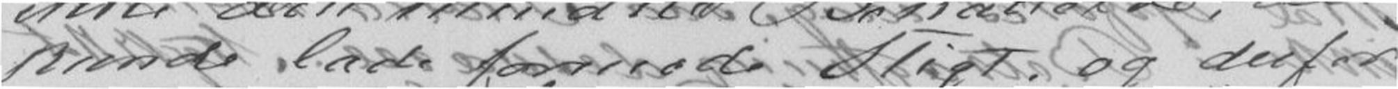kunde lade formode Sligt, og derfor
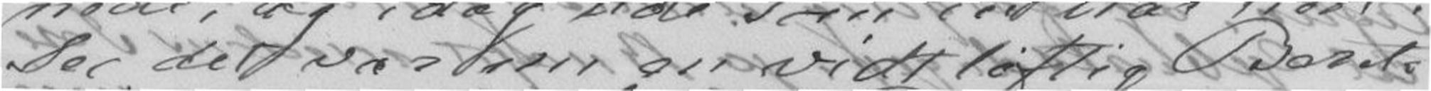See det var nu vidtløftig Beret-
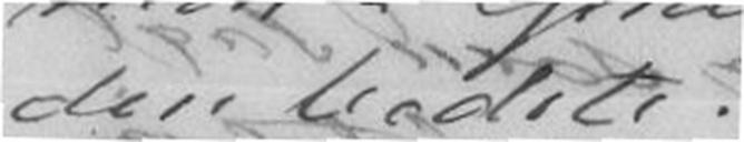den bedste. -
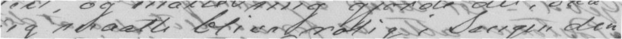jeg maatte blive rolig i Sengen den
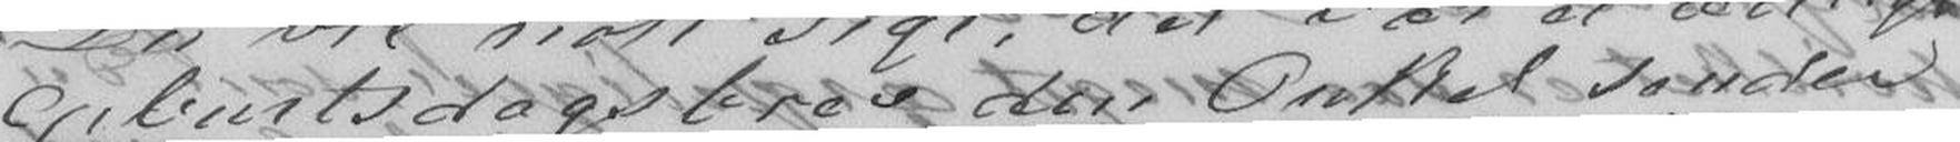Geburtsdagsbrev din Onkel sender
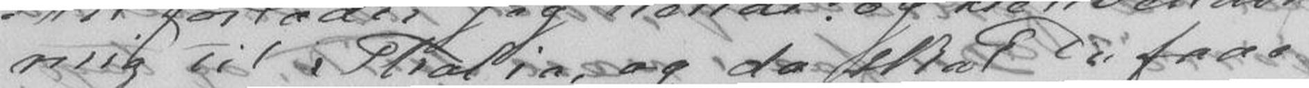mig til Tholia, og da skal Du faae
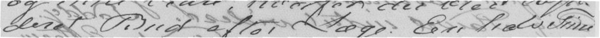deret Bud efter Læge. En halv Time
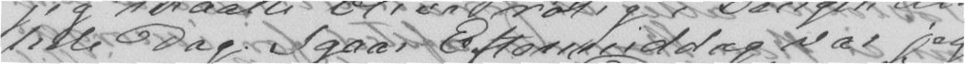hele Dag. Igaar Eftermiddag var jeg
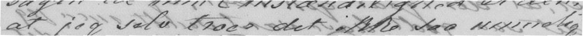at jeg selv troer det ikke saa umuelig
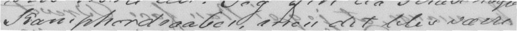Kamphordraaber, men det blev værre
